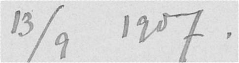13/9 1907.
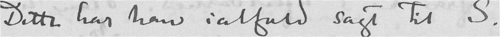Dette har han ialfald sagt til S.
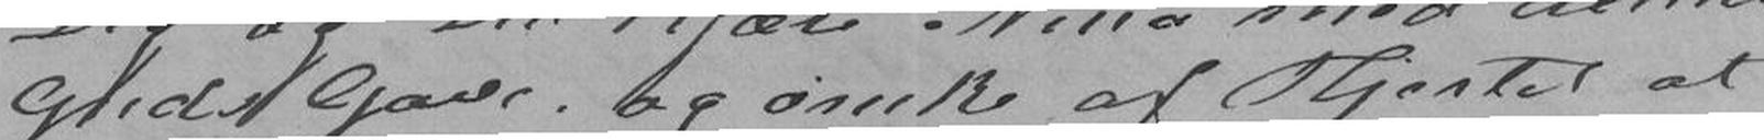Guds Gave, og ønske af Hjertet at
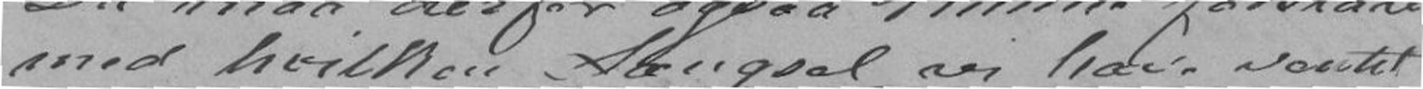med hvilken Længsel vi have ventet
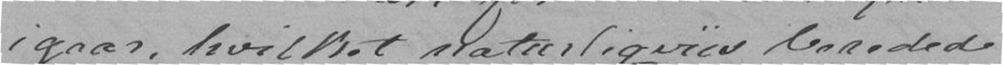igaar, hvilket naturligvis beredede
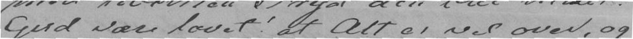Gud være lovet! at Alt er vel over, og
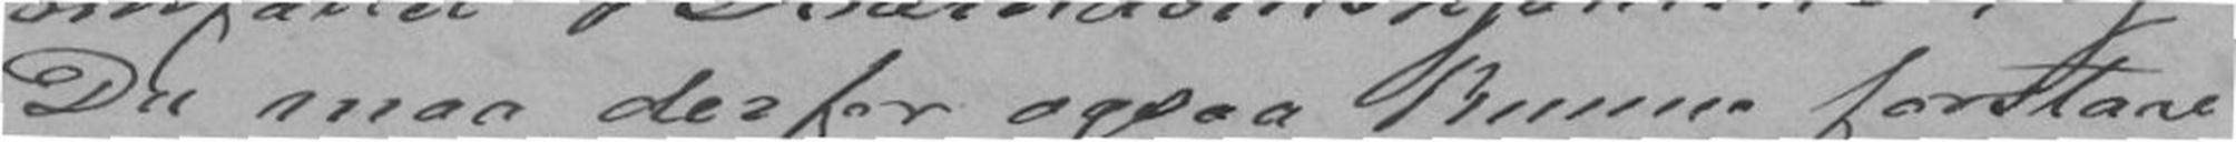Du maa derfor ogsaa kunne forstaae
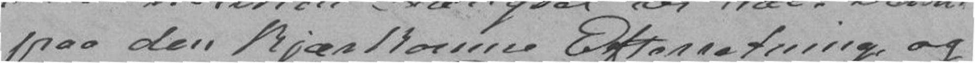paa den kjærekomne Efterretning og
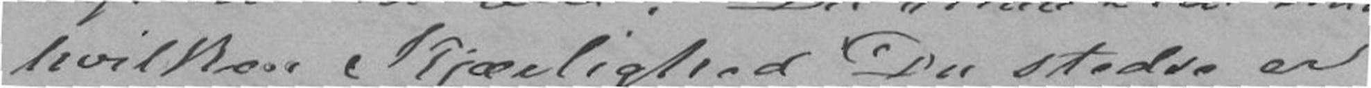hvilken Kjærlighed Du stedse er
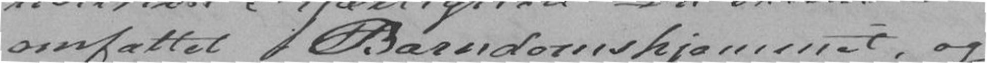omfattet i Barndomshjemmet, og
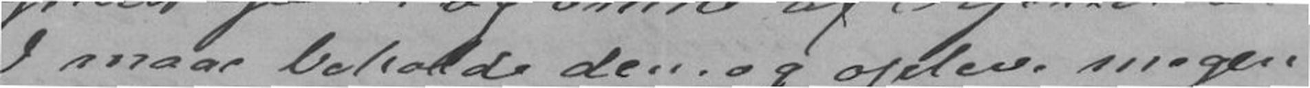I maae beholde den og opleve megen
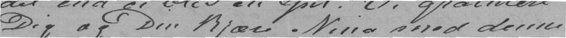Dig og Din kjære Nina med denne
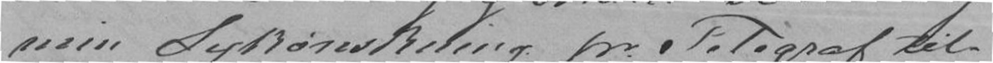min Lykønskning pr. Telegraf til-
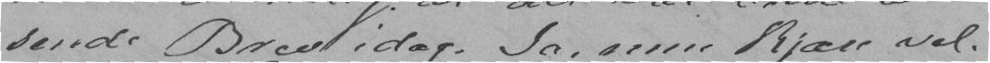sende Brev idag. Ja, min kjære vel-
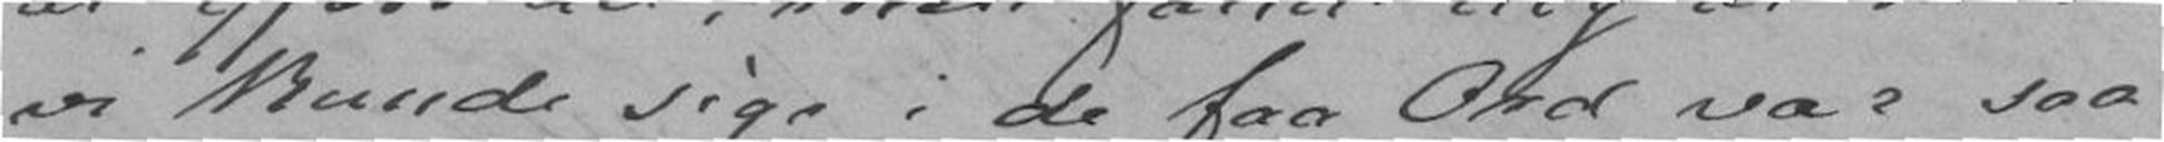vi kunde sige i de faa Ord var saa
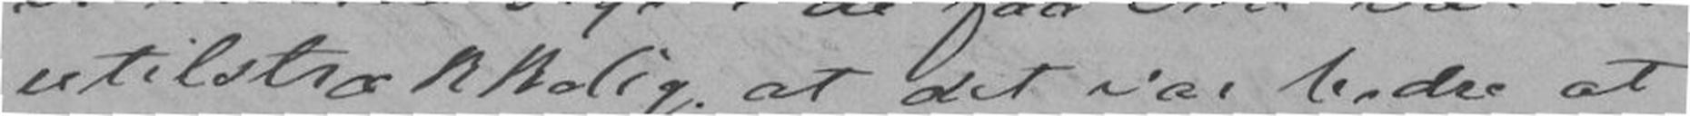utilstrækkelig at det var bedre at
